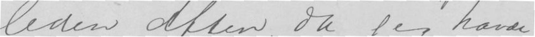leden Aften, da jeg havde
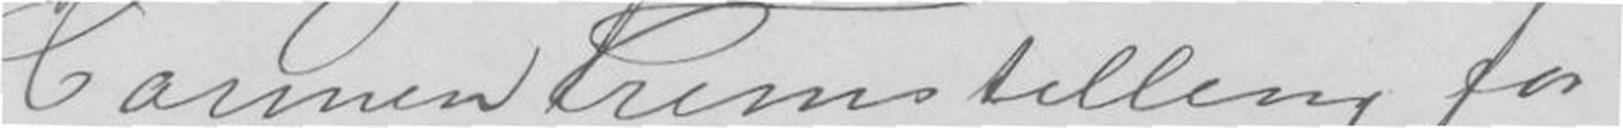Carmen Fremstilling for-
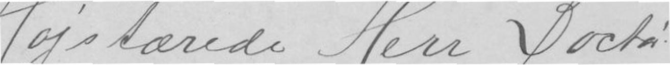Højstærede Herr Doctor!
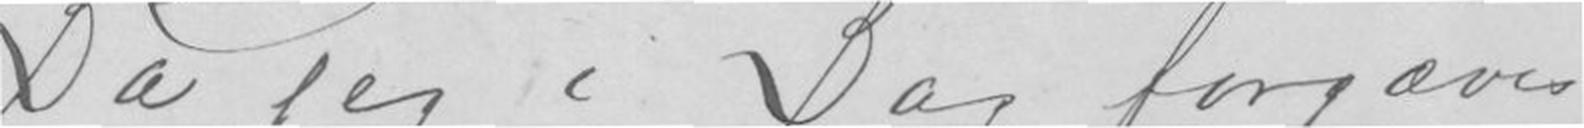Da jeg i Dag forgjæves
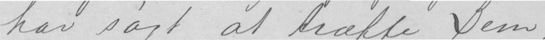har søgt at træffe Dem,
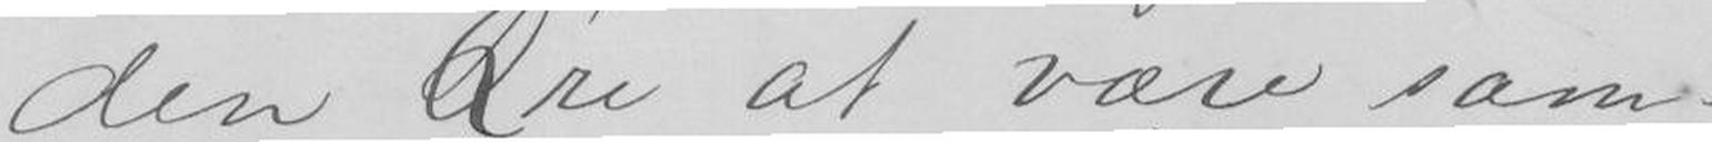den Ære at være sam-
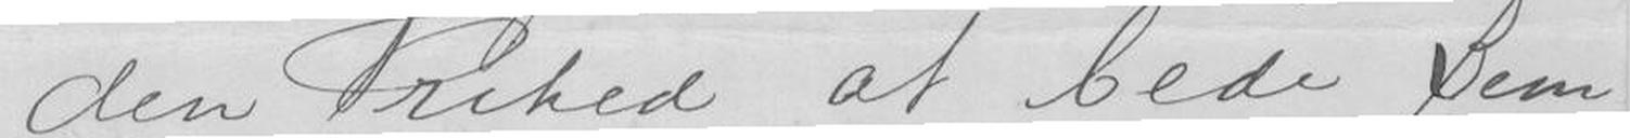den Frihed at bede Dem
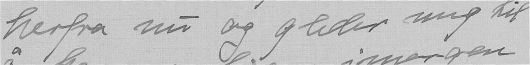herfra nu og gleder mig til
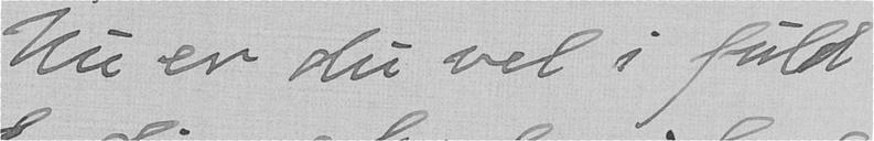Nu er du vel i fuld
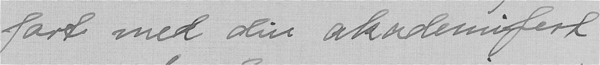fart med din akademifert
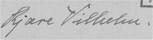Kjære Vilhelm.
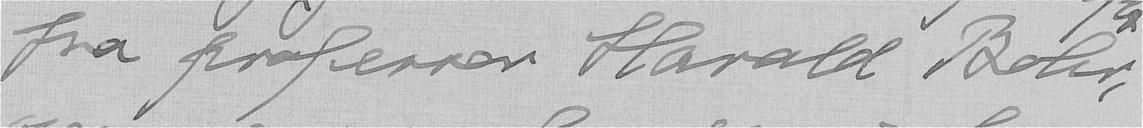fra professor Harald Bohr,
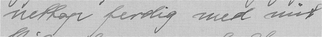nettop ferdig med mit
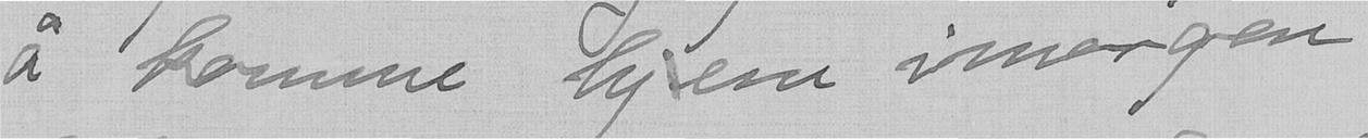å komme hjem imorgen
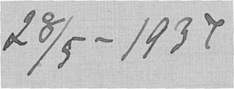28/5-1937
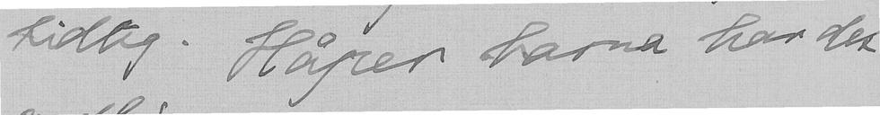tidlig. Håper barna har det
